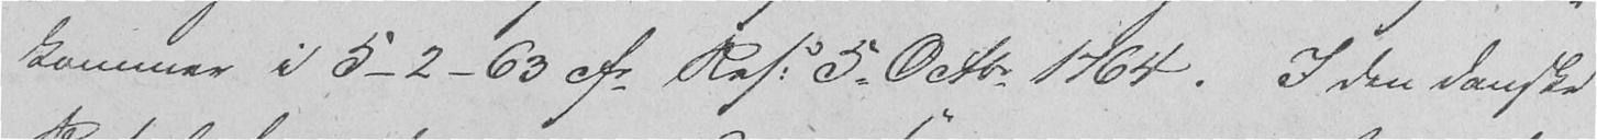kommer i 5-2-63 cfr Res. 5. Octbr 1764. I den danske
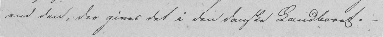end den, der gives det i den danske Landboret. -
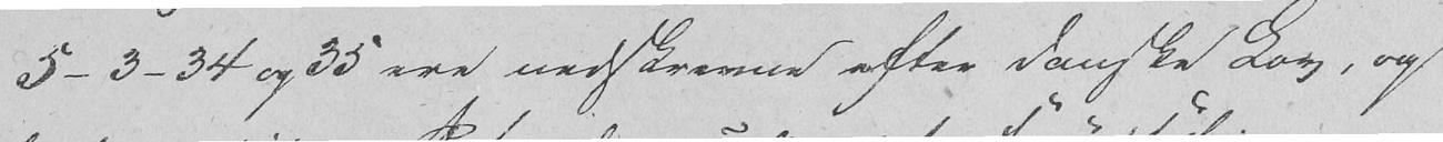5-3-34 og 35 ere nedskrevne efter danske Lov, og
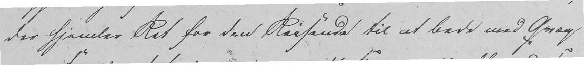der hjemler Ret for den Reisende til at bede med Qvæg
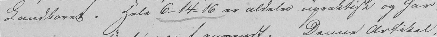Landboret. Hele 6-14-16 er aldeles upraktisk og har
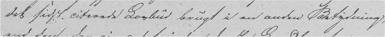det sidst citerede Lovbud brugt i en anden Betydning,
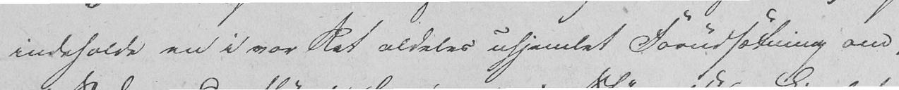indeholde en i vor Ret aldeles uhjemlet Forudsætning om,
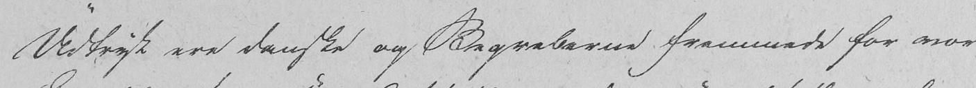Udtryk ere danske og Begreberne fremmede for vor
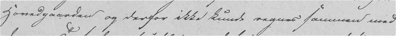Hovedgaarden og derfor ikke kunde regnes sammen med
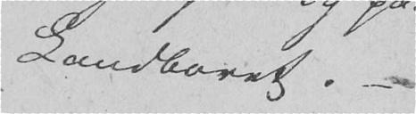Landboret. -
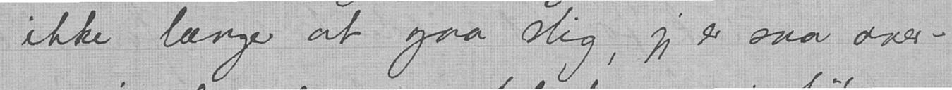ikke længer at gaa slig, jeg er saa over-
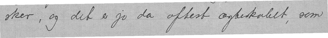sker, og det er jo da oftest ægteskabet, som
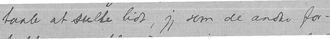taale at sulte lidt, jeg som de andre for-
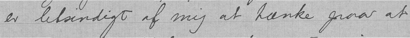er letsindigt af mig at tænke paa at
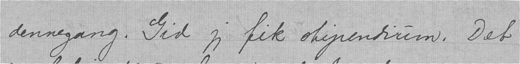dennegang. Gid jeg fik stipendium. Det
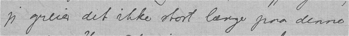jeg greier det ikke stort længer paa denne
